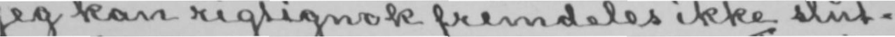Jeg kan rigtignok fremdeles ikke slut-
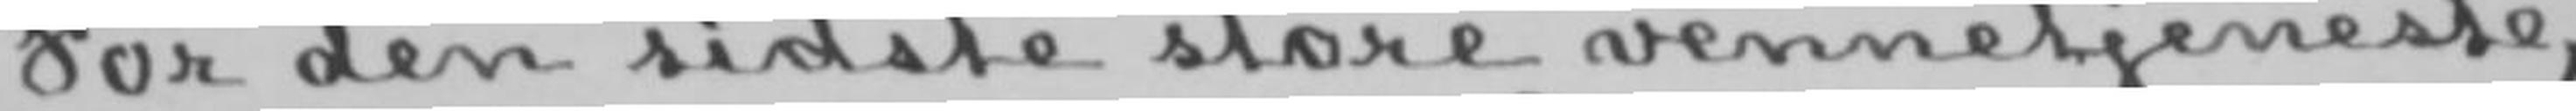For den sidste store vennetjeneste,
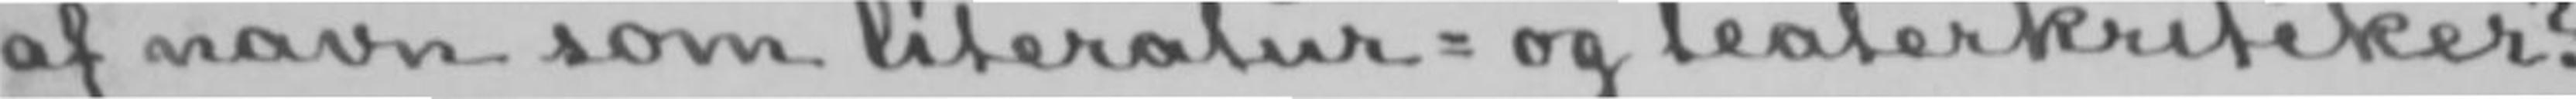af navn som literatur- og teaterkritiker?
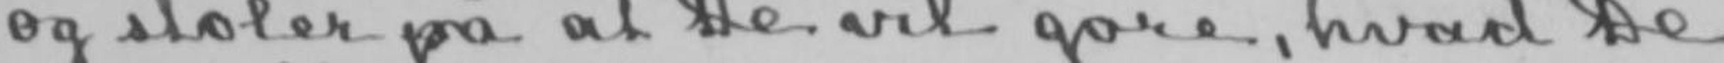og stoler på at De vil gøre, hvad De
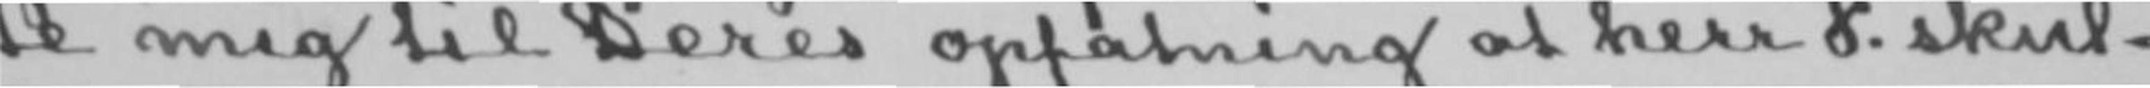te mig til Deres opfatning at herr F. skul-
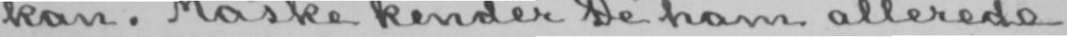kan. Måske kender De ham allerede
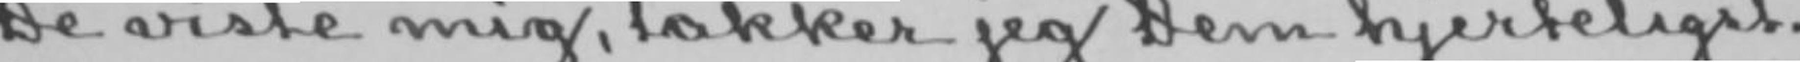De viste mig, takker jeg Dem hjerteligst.
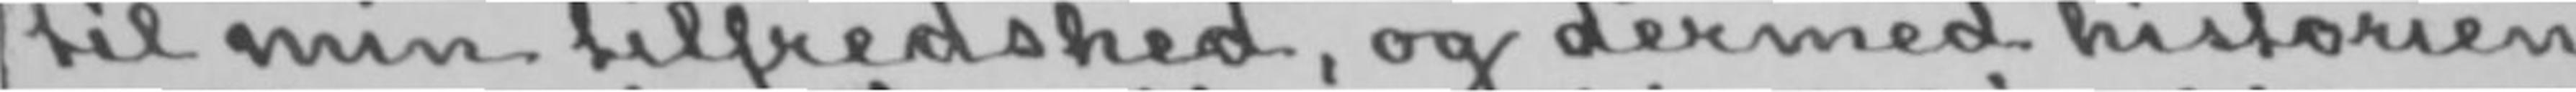til min tilfredshed, og dermed historien
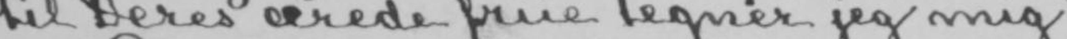til Deres ærede frue tegner jeg mig
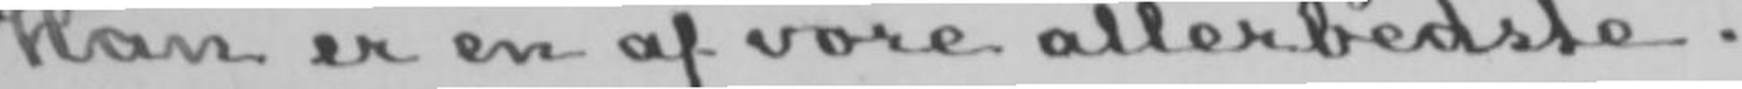Han er en af vore allerbedste.-
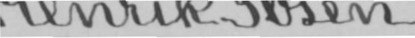Henrik Ibsen.
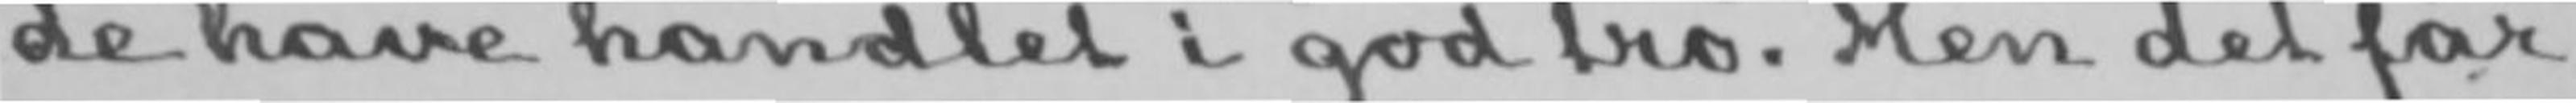de have handlet i god tro. Men det får
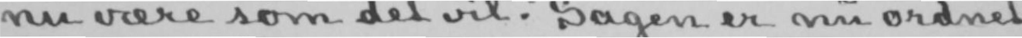nu være som det vil. Sagen er nu ordnet
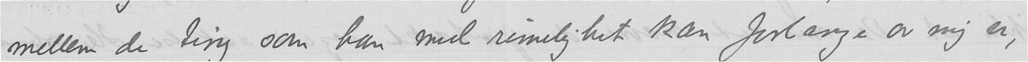mellem de ting som han med rimelighet kan forlange av mig er,
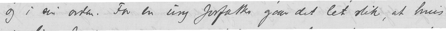og i sin orden. For en ung forfatter gaar det let slik, at hvis
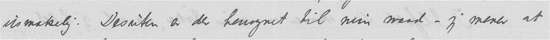usmakelig. Desuten er der hensynet til min mand – jeg mener at
